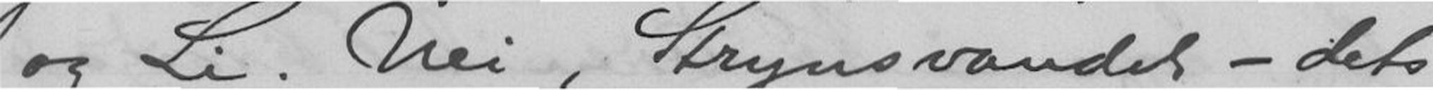og Li. Nei, Strynsvandet - dets
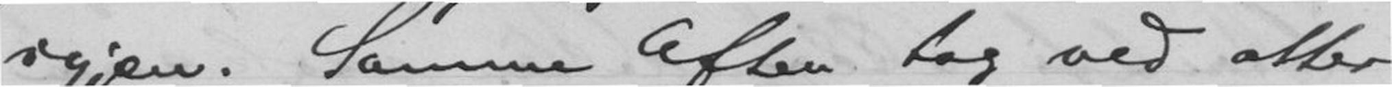igjen. Samme Aften tog ved atter
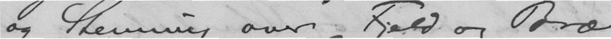og Stemning over Fjeld og Bræ
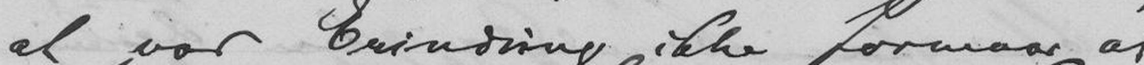at vor Erindring ikke formaar at
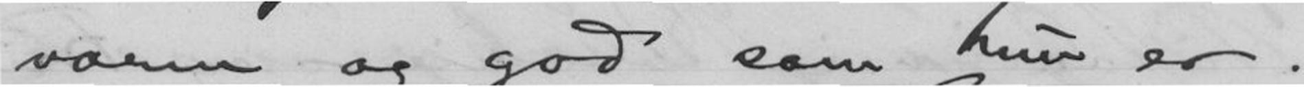varm og god som hun er.
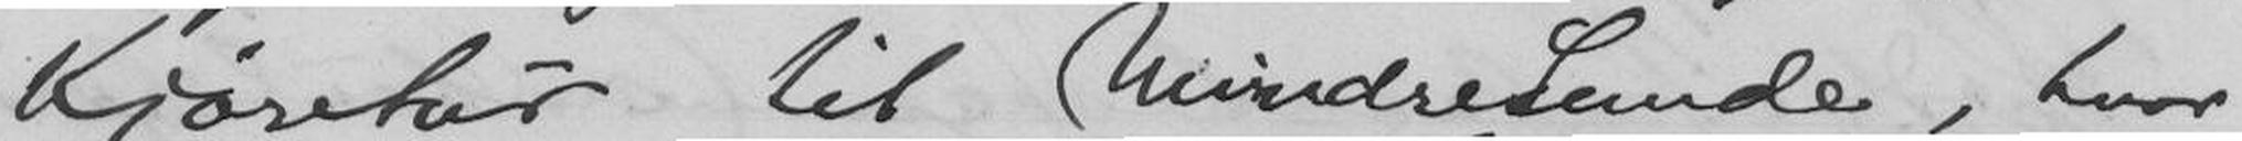kjøretur til Mindresunde, hvor
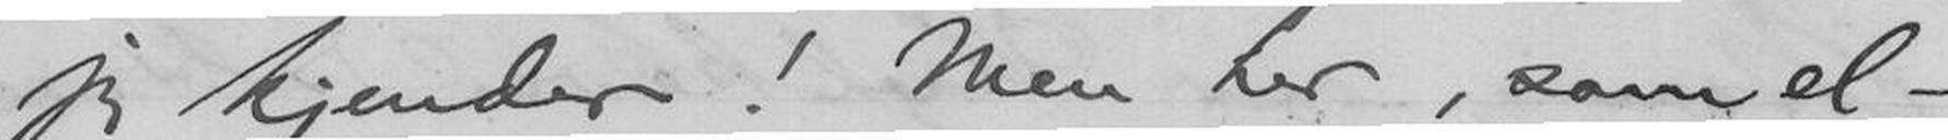jeg kjender! Men her, som el-
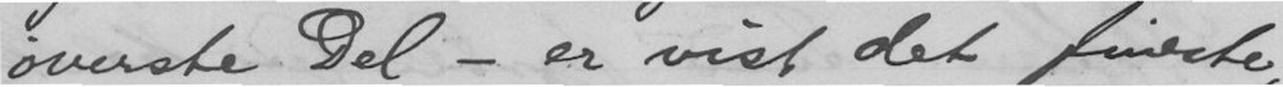øverste Del - er vist det fineste,
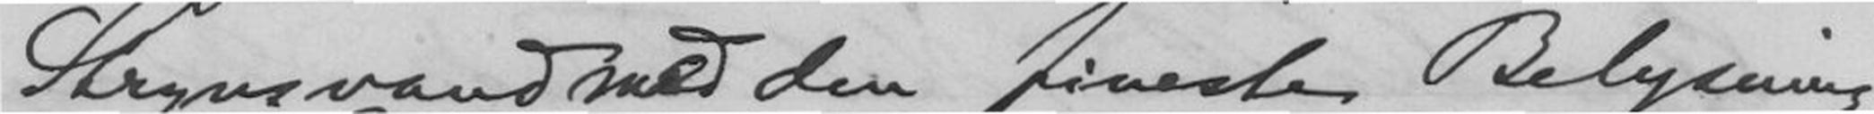Strynsvand med den fineste Belysning
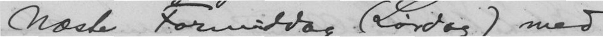Næste Formiddag (Lørdag) med
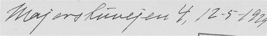Majorstuvejen 4, 12-5-1929
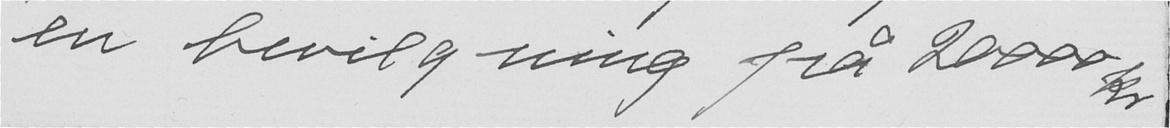en bevilgning fra 20000 kr
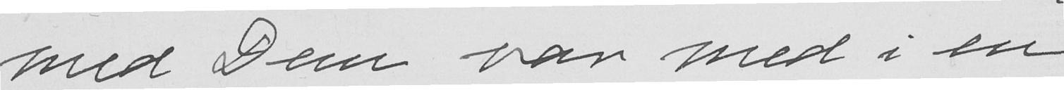med Dem var med i en
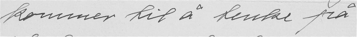kommer til å tenke på
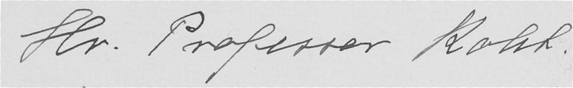Hr. Professer Koht.
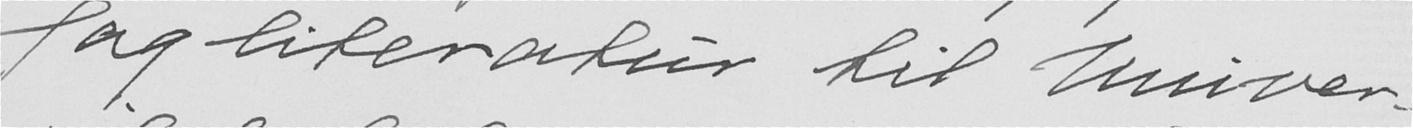fagliteratur til Univer-
